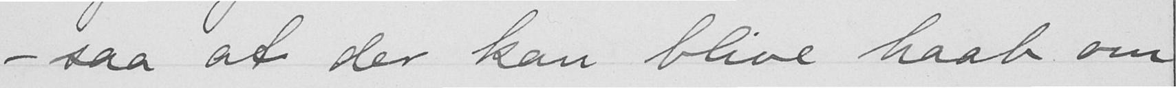- saa at der kan blive haab om
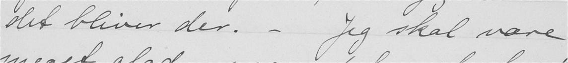det bliver der. - Jeg skal være
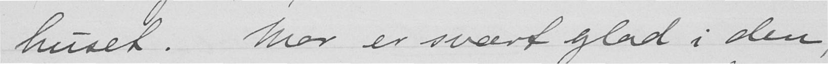huset. Mor er svært glad i den,
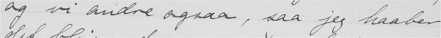og vi andre ogsaa, saa jeg haaber
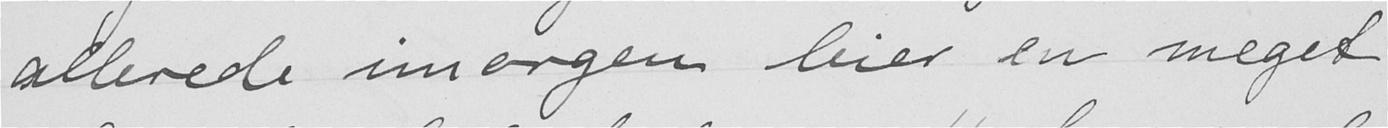allerede imorgen leier en meget
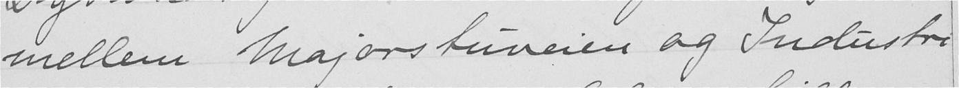mellem Majorstuveien og Industri-
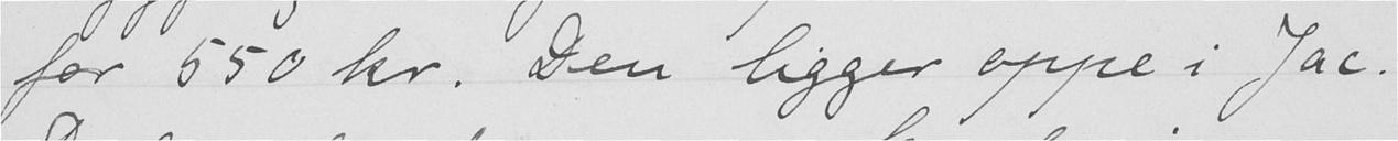for 550 kr. Den ligger oppe i Jac.
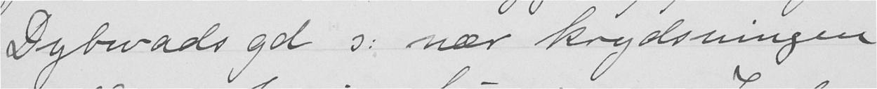Dybvads gd: nær krydsningen
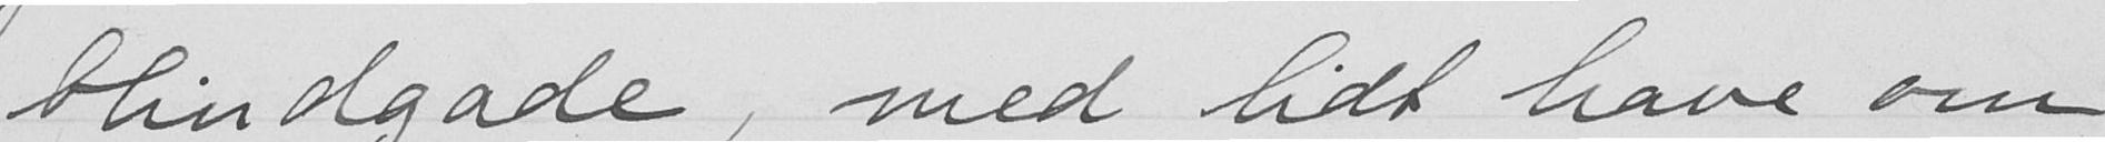blindgade, med lidt have om
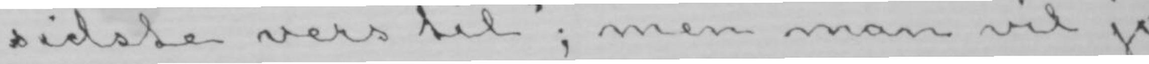sidste vers til»; men man vil jo
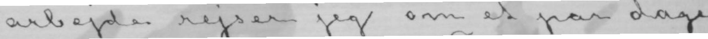arbejde rejser jeg om et par dage
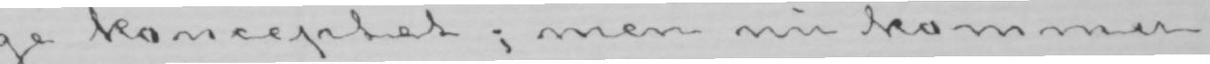ge konceptet; men nu kommer
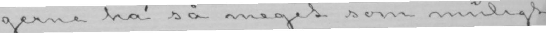gerne ha&rapo; så meget som muligt
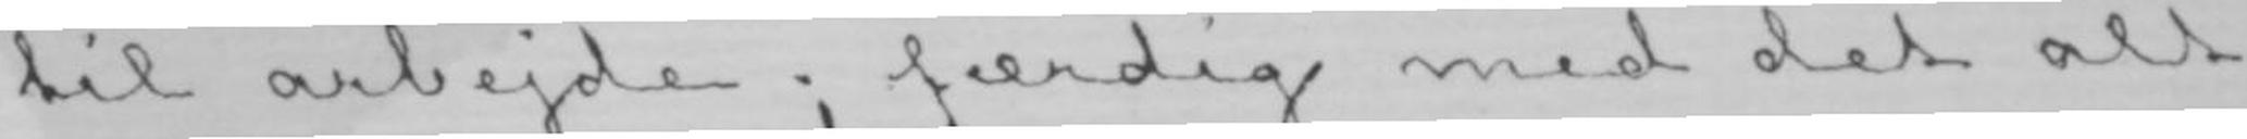til arbejde; færdig med det alt
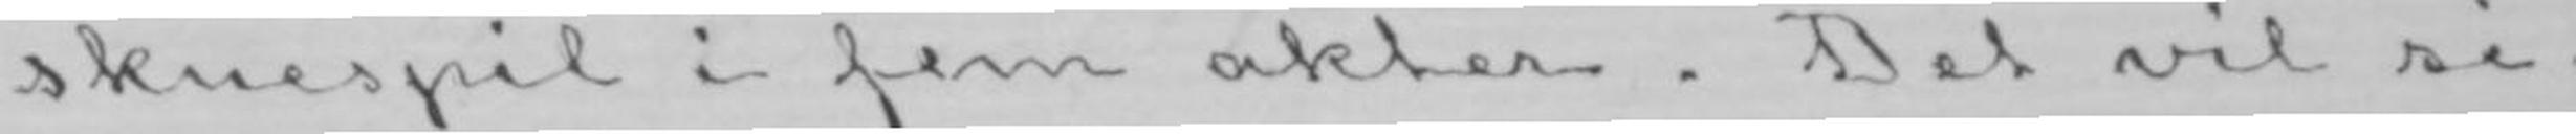skuespil i fem akter. Det vil si-
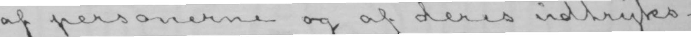af personerne og af deres udtryks-
